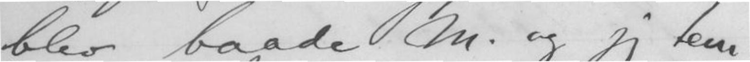ningens
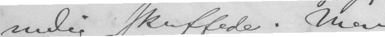melig skuffede. Men
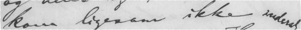kom ligesom ikke inderst
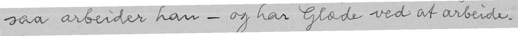saa arbeider han - og har Glæde ved at arbeide.
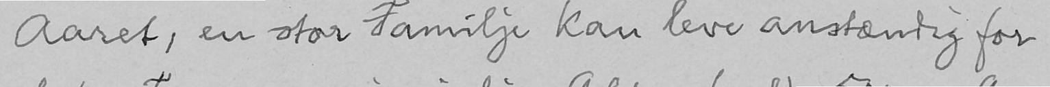Aaret, en stor Familje kan leve anstændig for
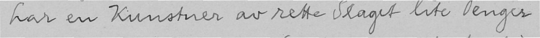har en Kunstner av rette Slaget lite Penger
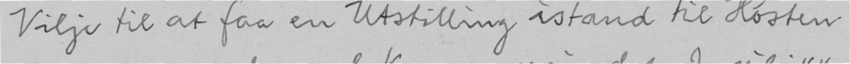Vilje til at faa en Utstilling istand til Høsten
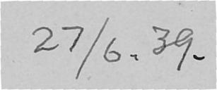27/6. 39.
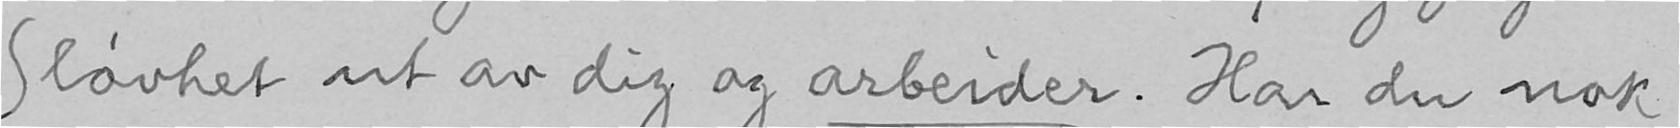Sløvhet ut av dig og arbeider. Har du nok
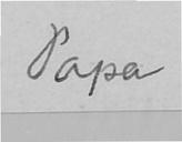Papa
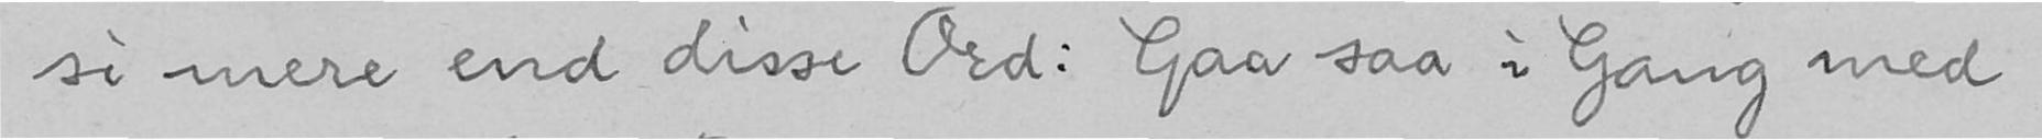si mere end disse Ord: Gaa saa i Gang med
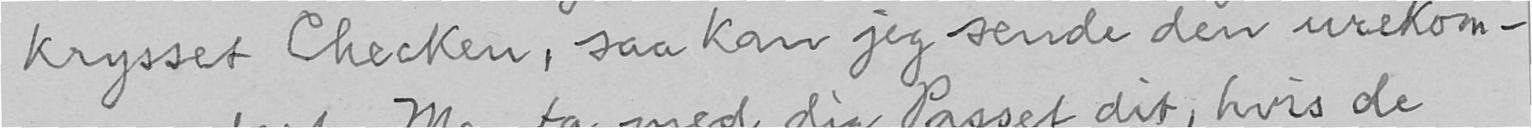krysset Checken, saa kan jeg sende den urekom-
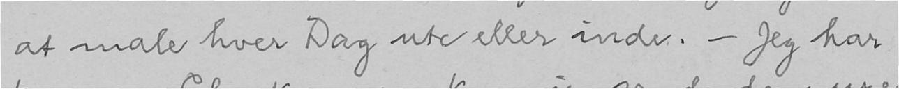at male hver Dag ute eller inde. - Jeg har
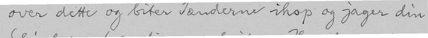over dette og biter Tænderne ihop og jager din
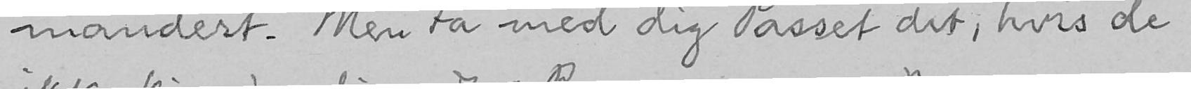mandert. Men ta med dig Passet dit, hvis de
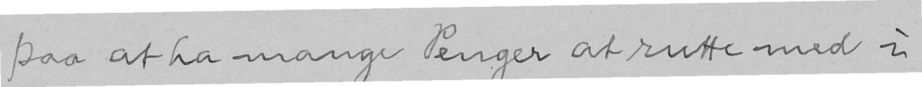paa at ha mange Penger at rutte med i
